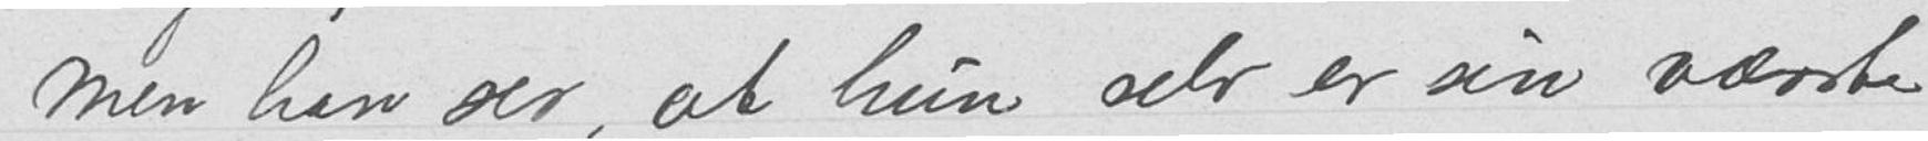men han ser, at hun selv er sin værste
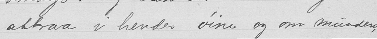attraa i hendes øine og om munden,
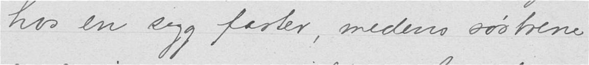hos en syg faster, medens søstrene
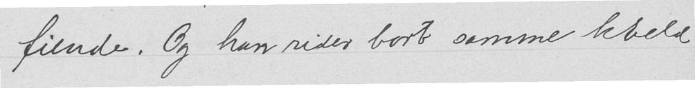fiende. Og han reiser bort samme kveld
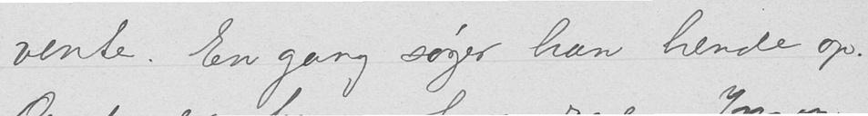vente. En gang søger han hende op.
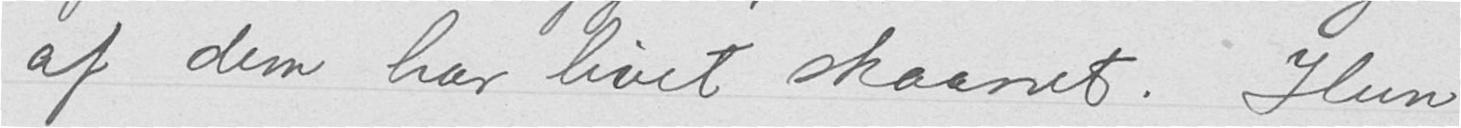af dem har livet skaanet. Hun
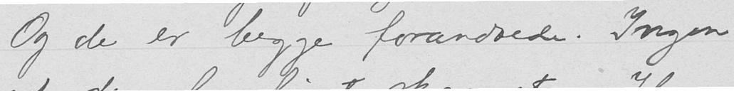Og de er begge forandrede. Ingen
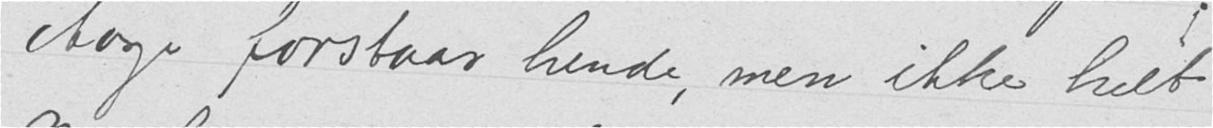Aage forstaar hende, men ikke helt
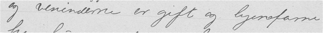og veninderne er gift og hjemefarne
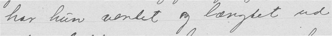har hun ventet og længtet ud
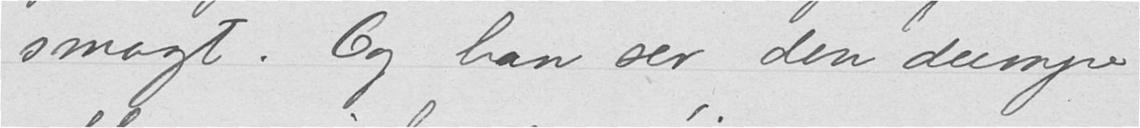smaagt. Og han ser den dumpe
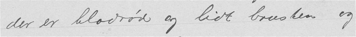der er blodrød og lidt brusten og
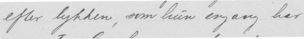efter lykken, som hun engang har
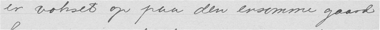er vokset op paa den ensomme gaard
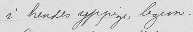i hendes yppige legem.
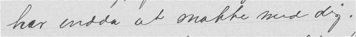har endda at snakke med dig.
